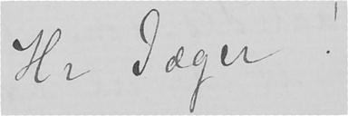Hr Jæger!
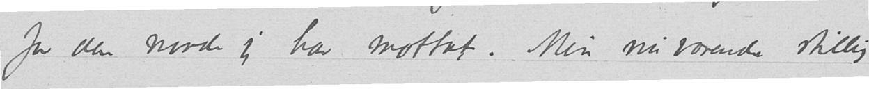for den naade jeg har mottat. Min nuværende stilling
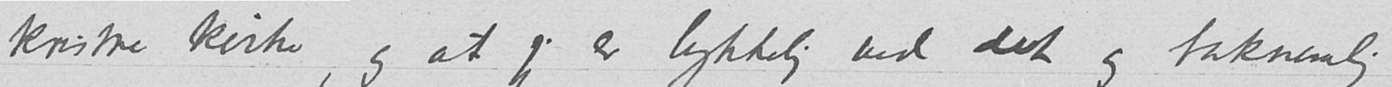kristne kirke, og at jeg er lykkelig ved det og taknemlig
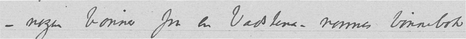– nogen bønner fra en Vadstena-nonnes bønnebok
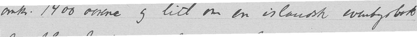omkr. 1400 aarene og litt om en islandsk eventyrbok
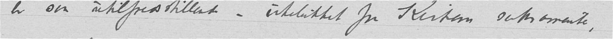er saa utilfredsstillende – utelukket fra Kirkens sakramenter,
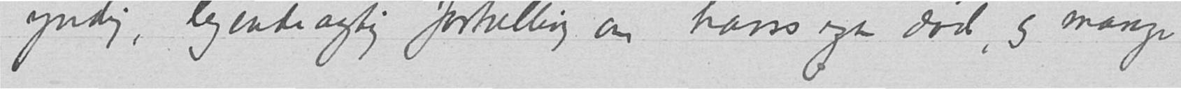yndig, legendeagtig fortælling om hans egen død, og mange
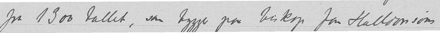fra 1300 tallet, som bygger paa biskop Jon Halldorsons
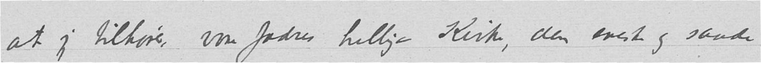at jeg tilhører vore fædres hellige Kirke, den eneste og sande
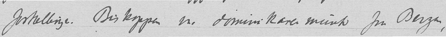fortellinger.  Biskoppen var dominikanermunk fra Bergen,
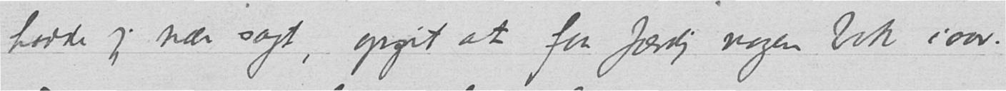hadde jeg nær sagt, opgit at faa færdig nogen bok i aar.
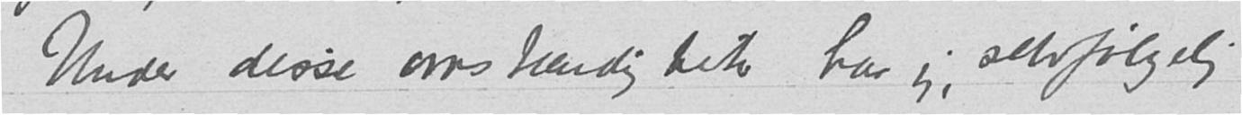Under disse omstændigheter har jeg, selvfølgelig
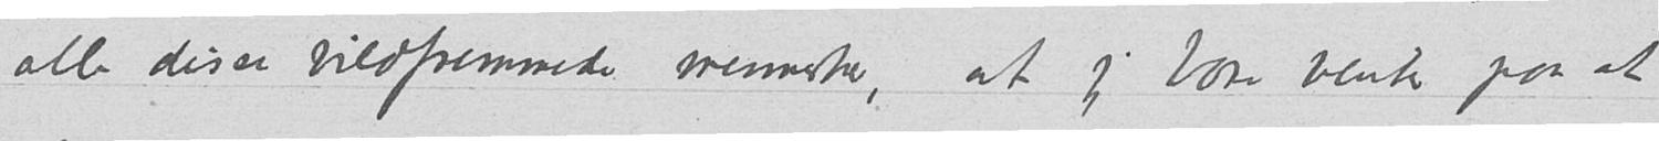alle disse vildfremmede mennesker, at jeg bare venter paa at
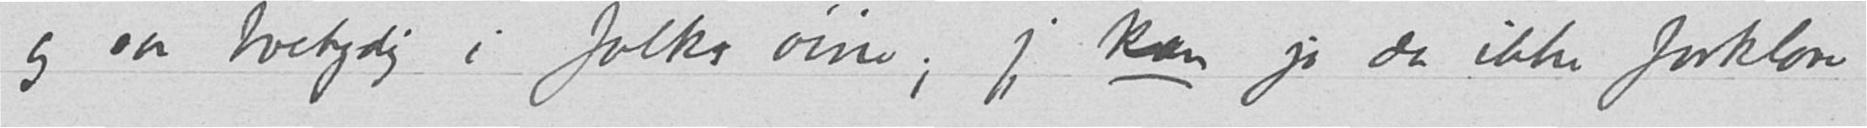og saa tvetydig i folks øine; jeg kan jo da ikke forklare
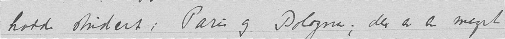hadde studert i Paris og Bologna; der er en meget
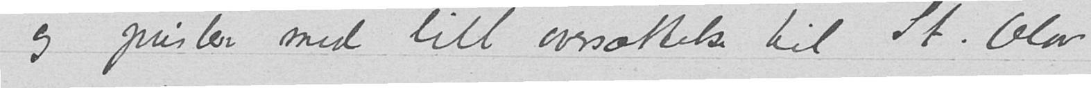og pusler med litt oversættelse til St. Olav
